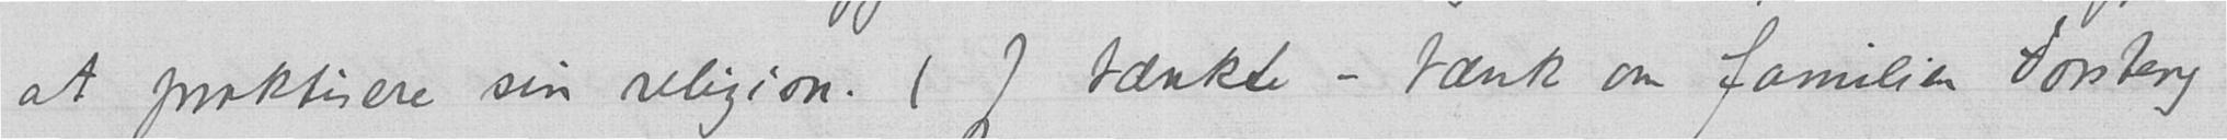at praktisere sin religion. (Jeg tænkte – tænk om familien Forsberg
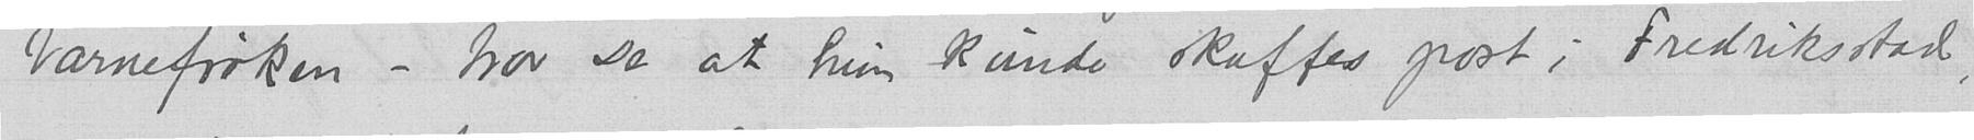barnefrøken – tror De at hun kunde skaffes post i Fredriksstad,
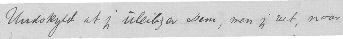Undskyld at jeg uleiliger Dem, men jeg vet, naar
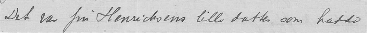Det var fru Henrichsens lille datter som hadde
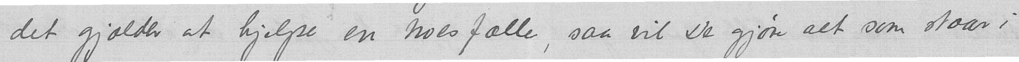det gjælder at hjelpe en troesfælle, saa vil De gjøre alt som staar i
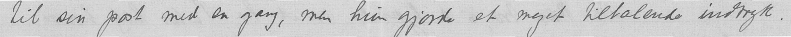til sin post med en gang, men hun gjorde et meget tiltalende indtryk.
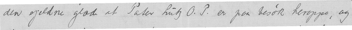den sjeldne glæde at Pater Lutz O.P. er paa besøk heroppe, og
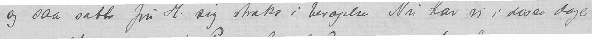og saa satte fru H. sig straks i bevægelse. Nu har vi i disse dage
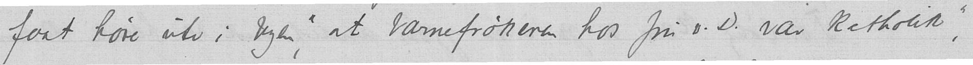faat høre ute i byen "at barnefrøkenen hos fru v. D. var katholik",
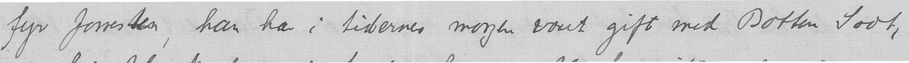fyr forresten, han har i tidernes morgen været gift med Botten Soot,
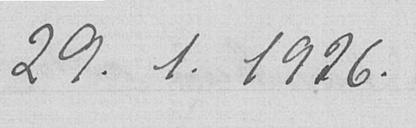29.1.1926
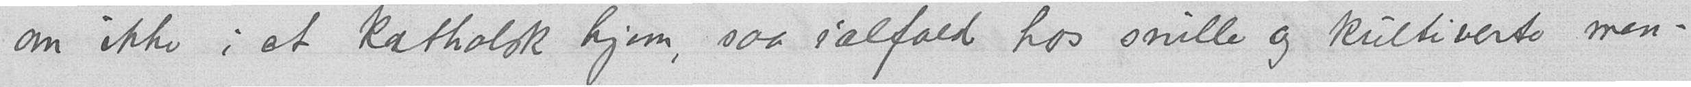om ikke i et katholsk hjem, saa ialfald hos snille og kultiverte men-
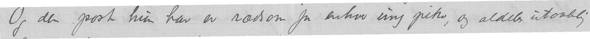Og den post hun har er rædsom for enhver ung pike, og aldeles utaalelig
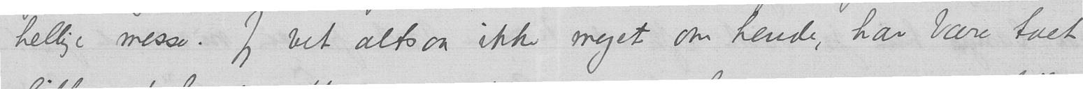hellige messe. Jeg vet altsaa ikke meget om hende, har bare talt
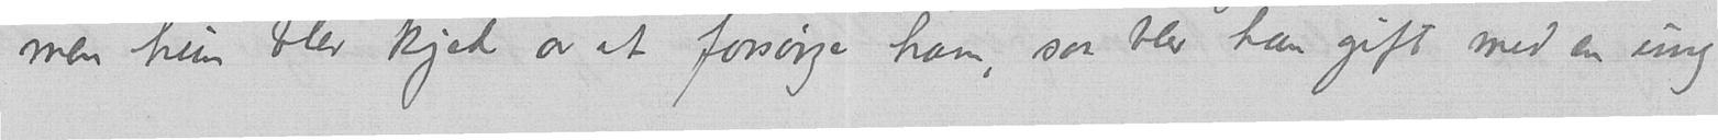men hun blev kjed av at forsørge ham, saa blev han gift med en ung
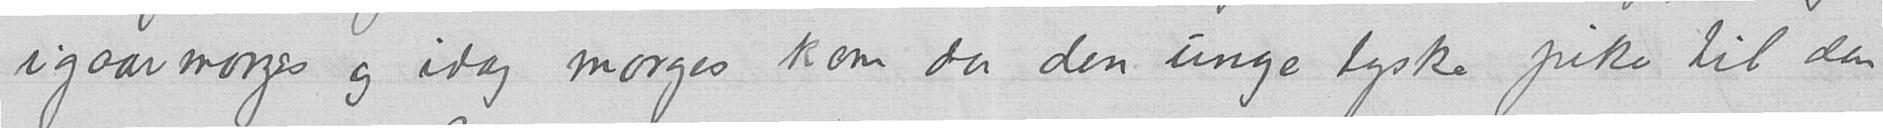igaarmorges og idag morges kom da den unge tyske pike til den
